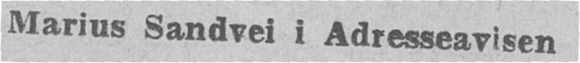Marius Sandvei i Adresseavisen
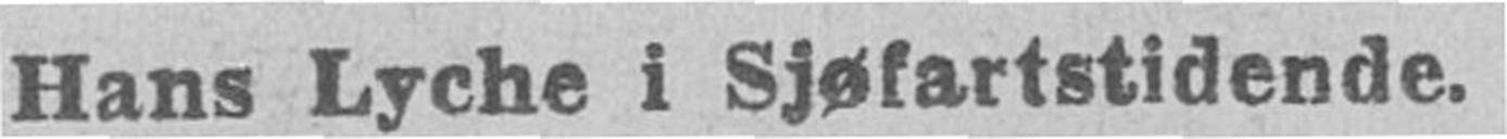Hans Lyche i Sjøfartstidende.
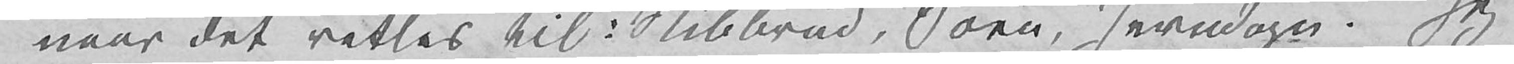naar det rettes til: Skibbrud, Søen, Jevndøgn. Jeg
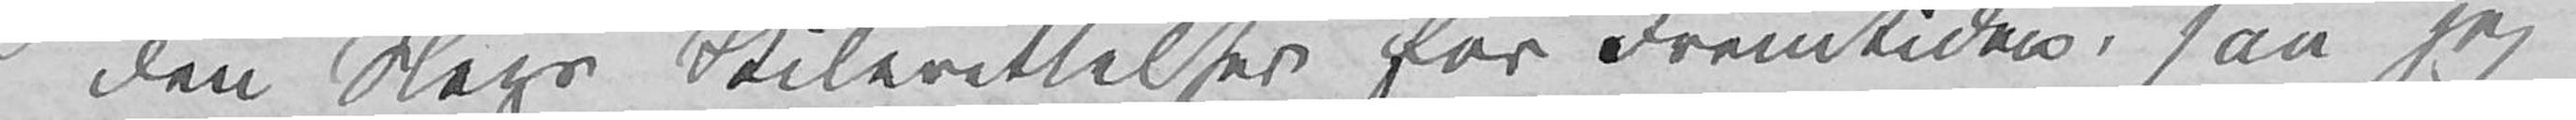den Slags Stilerettelser for Fremtiden, saa jeg
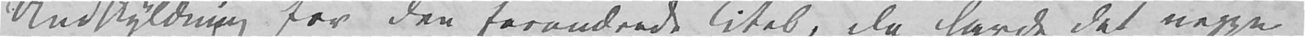Undskyldning for den forandrede Titel, da havde det neppe
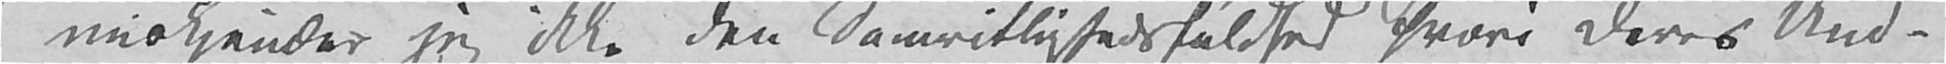miskjender jeg ikke den Samvittighedsfuldhed hvori Deres Und¬
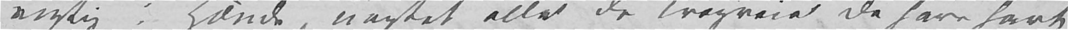rigtig i Hænde, uagtet alle de Krogveie de have havt
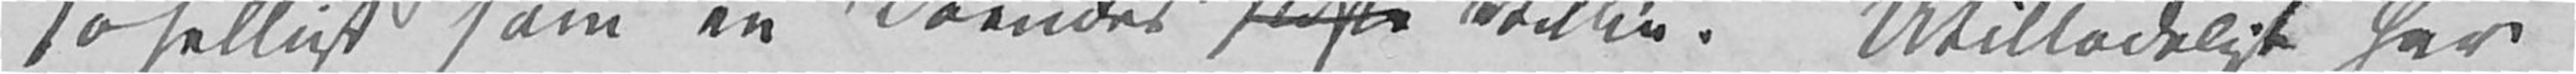ja helligt som en Døendes sidste Villie. Utilladeligt har
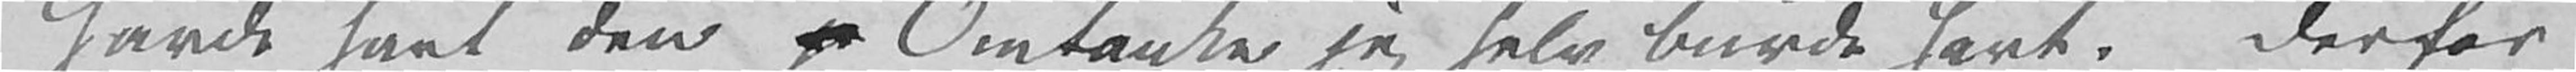havde havt den <gap /></del> Omtanke jeg selv burde havt. Derfor
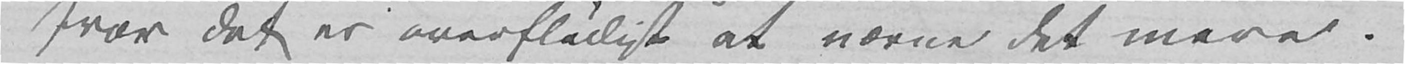tror det er overflødigt at nævne det mere.
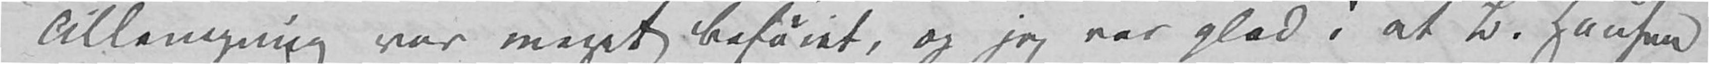Tillempning var meget beføiet, og jeg var glad i at B. Hansen
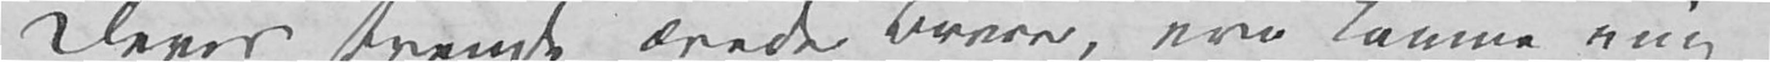Deres tvende ærede Breve, ere komne mig
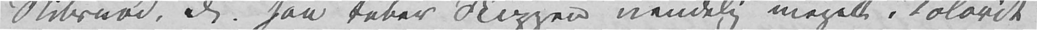Skibsnød, etc. saa taber Skizzen uendelig meget i Kolorit
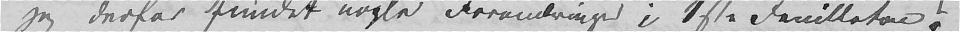jeg derfor fundet nogle Forandringer i 1ste Feuilleton,
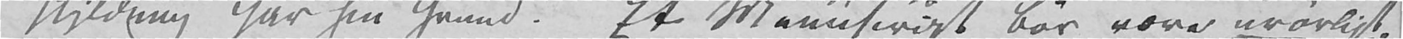skyldning har sin Grund. Et Manuscript bør være urørligt,
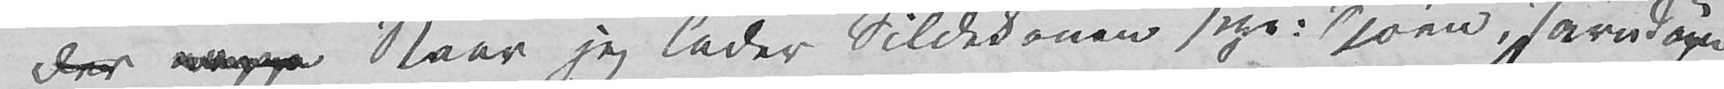der neppe Naar jeg lader Sildekonen sige: Sjøen, Javndøgn
